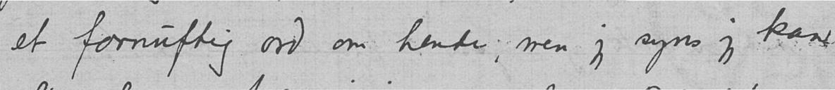et fornuftig ord om hende, men jeg syns jeg kan
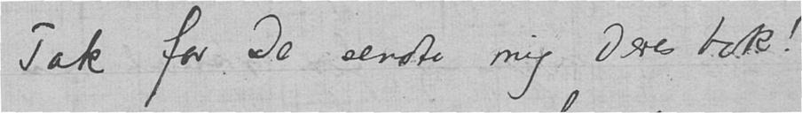Tak for De sendte mig. Deres bok!
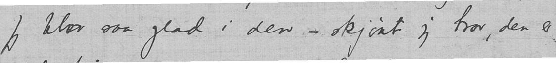Jeg blæv saa glad i den - skjønt jeg tror, den er
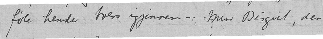føle hende tvers igjennem –. Men Birgit, den
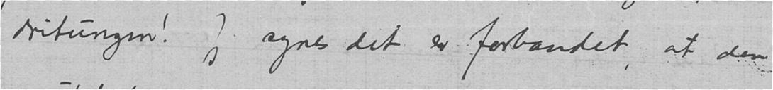dritungen! Jeg synes det er forbandet, at den
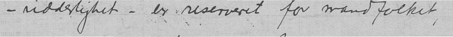– ridderlighet – er reserveret for mandfolket,
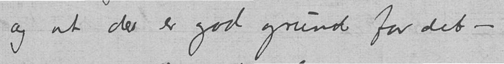og at der er god grund for det – .
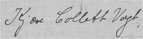Kjære Collett Vogt,
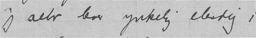Jeg selv lever ynkelig elendig i
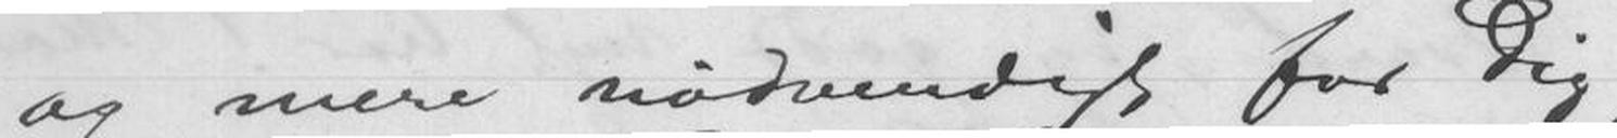og mere nødvendigt for Dig,
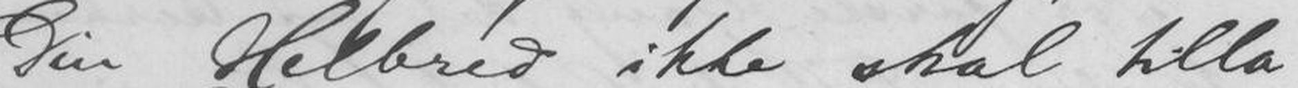Din Helbred ikke skal tilla-
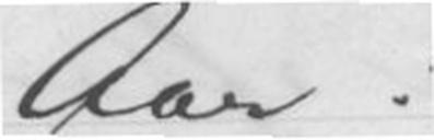Aar!
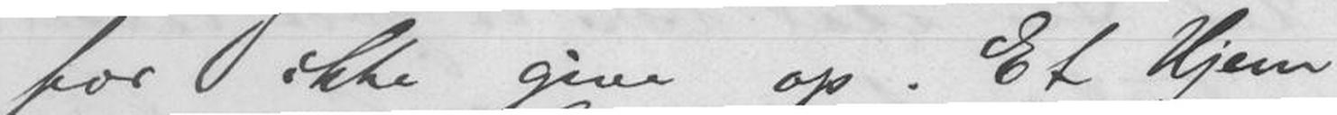for ikke give op. Et Hjem
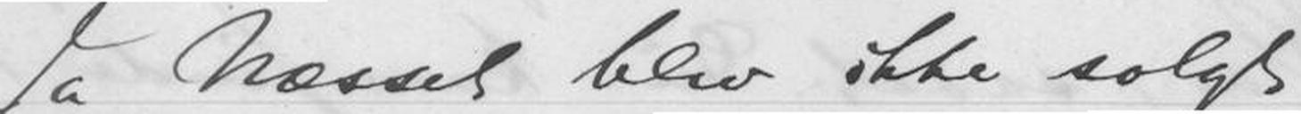Ja Næsset blev ikke solgt
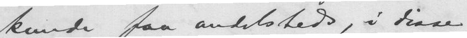kunde faa andetsteds, i disse
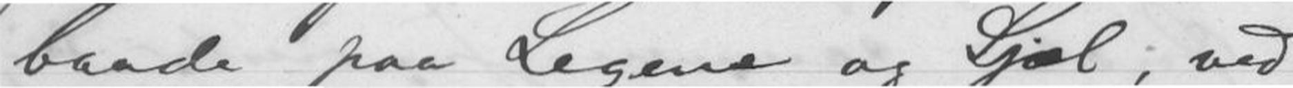baade paa Legeme og Sjæl; ved
